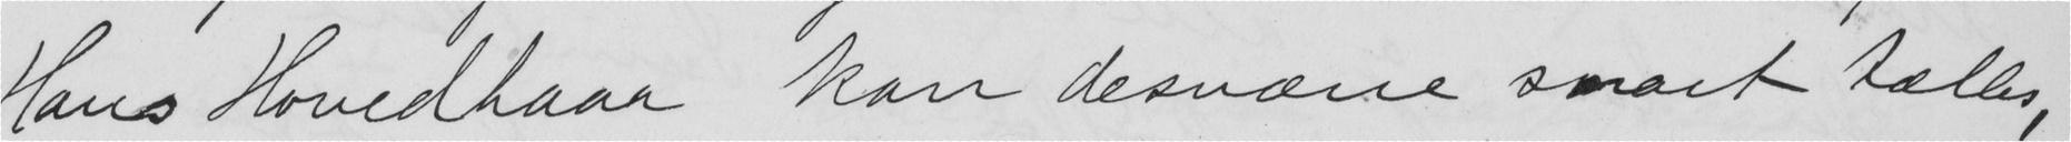Hans Hovedhaar kan desværre snart tælles,
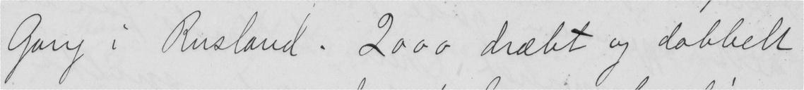Gang i Rusland. 2000 dræbt og dobbelt
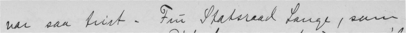var saa trist. Fru Statsraad Lange, som
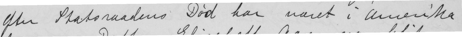efter Statsraadens Død har været i Amerika
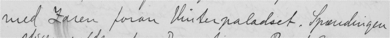med Zaren foran Vinterpaladset. Spændingen
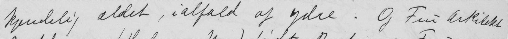kjendelig ældet, ialfald af ydre. Og Fru Arkitekt
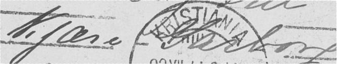Kjære Garborg
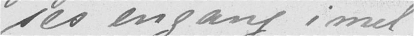ses engang i mel-
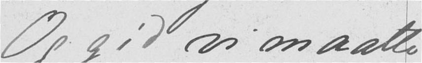Og gid vi maatte
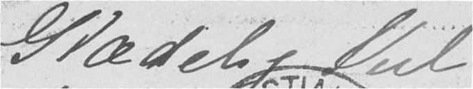Glædelig Jul
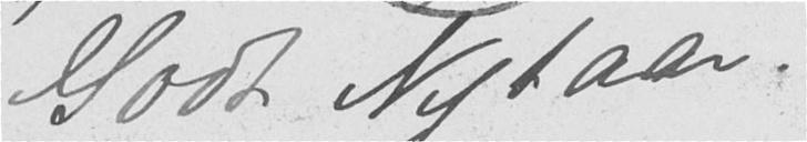Godt Nytaar!
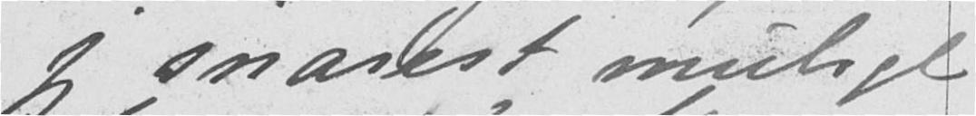jeg snarest muligt
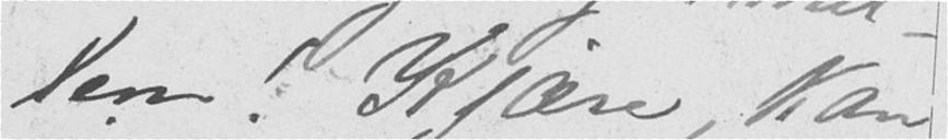lem. Kjære, kan
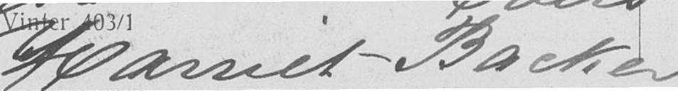Harriet Backer
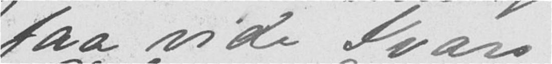faa vide Ivars
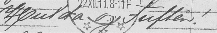Hulda og Tuften!
